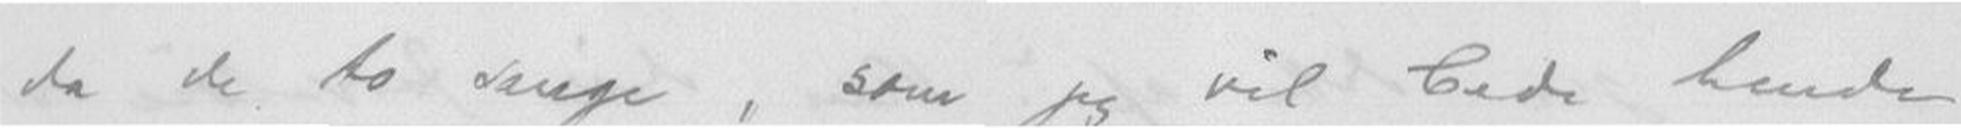da de to sange, som jeg vil bede hende
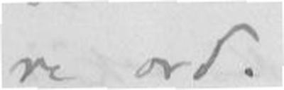re ord.
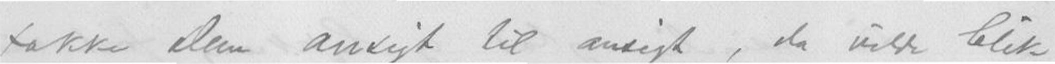takke Dem ansigt til ansigt, da vilde blik
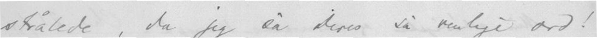strålede, da jeg så Deres så venlige ord!
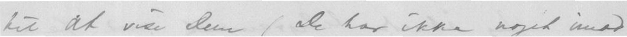til at vise Dem (De har ikke noget imod
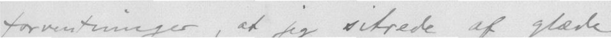forventninger, at jeg sitrede af glæde
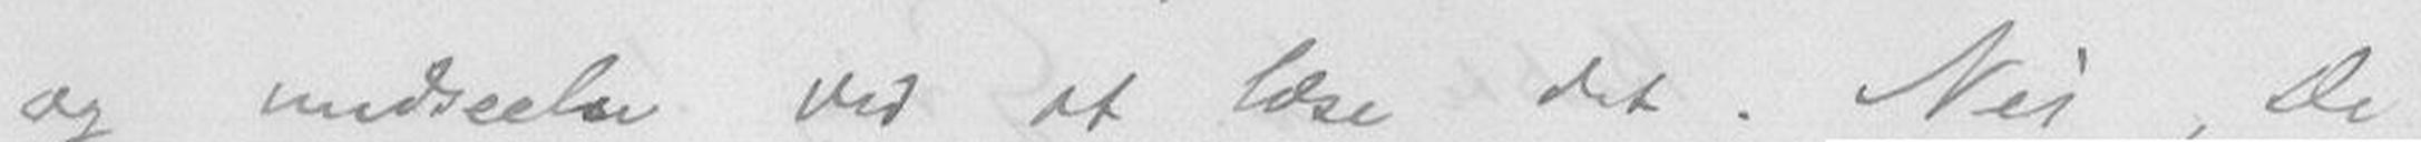og medseelse ved at læse det. Nei, De
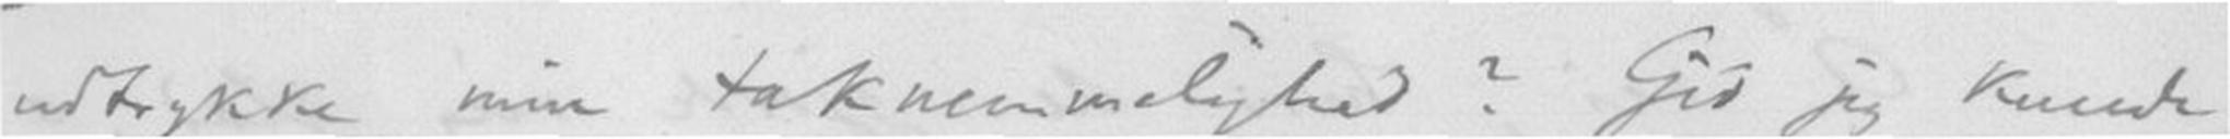udtrykke min taknemlighed? Gid jeg kunde
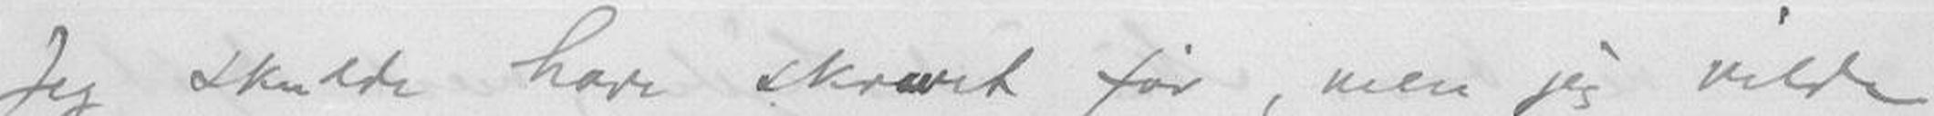Jeg skulde have skrevet før, men jeg vilde
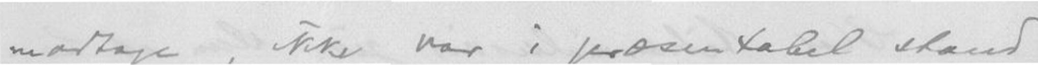modtage, ikke var i præsentabel stand
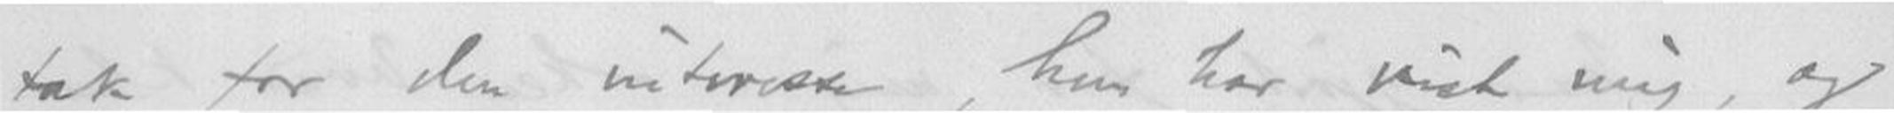tak for den intresse, hun har vist mig, og
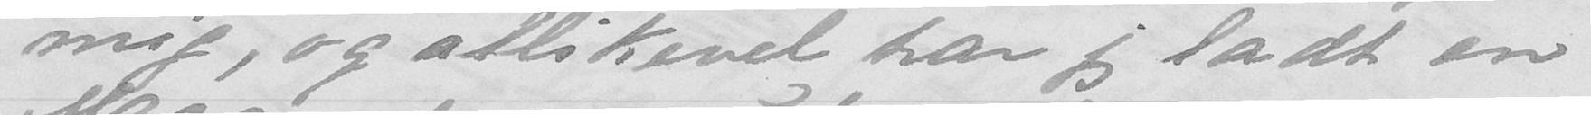mig, og allikevel har jeg ladt en
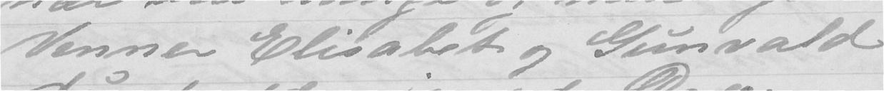Venner Elisabet og Gunvald
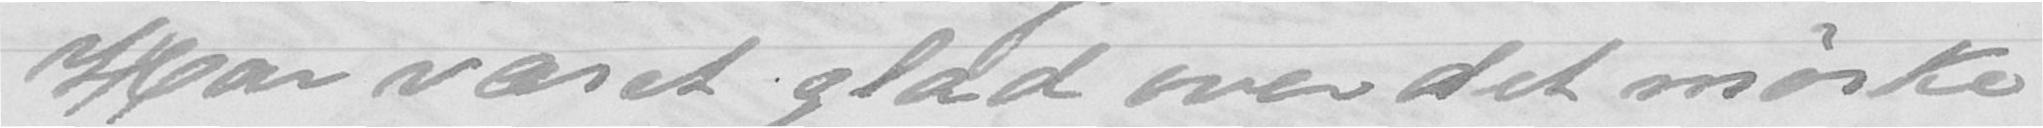Har været glad over det mørke
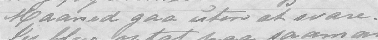Maaned gaa uten at svare.
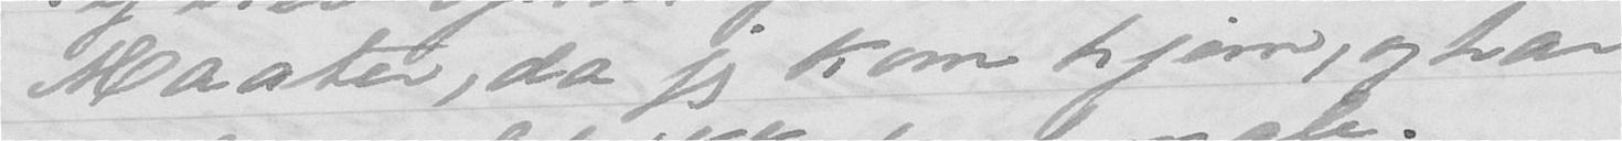Maater, da jeg kom hjem, og har
